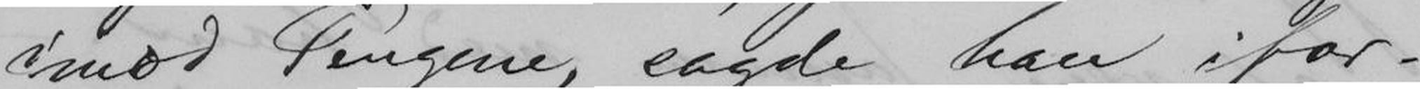imod Pengene, sagde ham ifor-
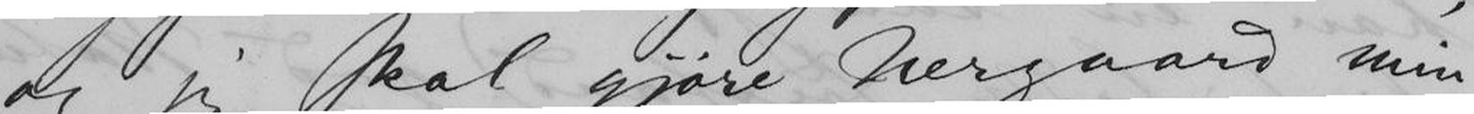og jeg skal gjøre Nergaard min
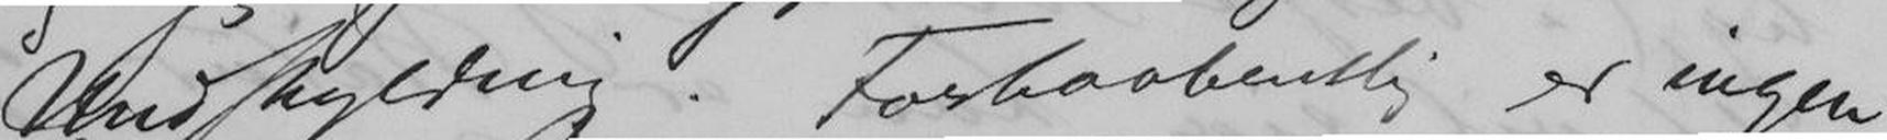Undskylding. Forhaabentlig er ingen
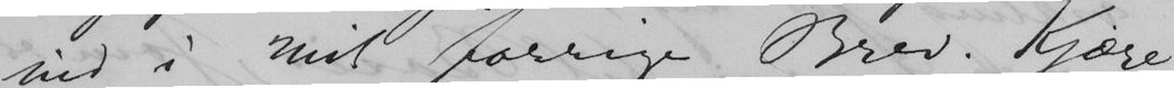ind i mit forrige Brev. Kjære
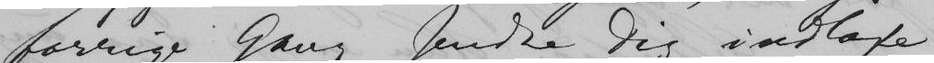forrige Gang sendte Dig indlagte
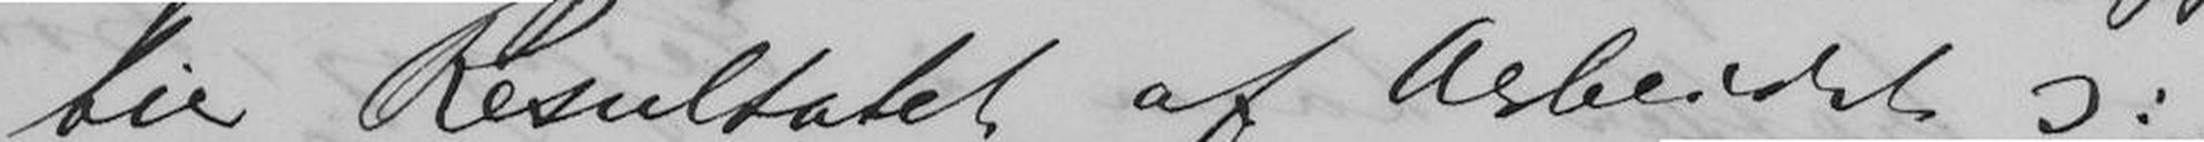bie Resultatet af Arbeidet ):
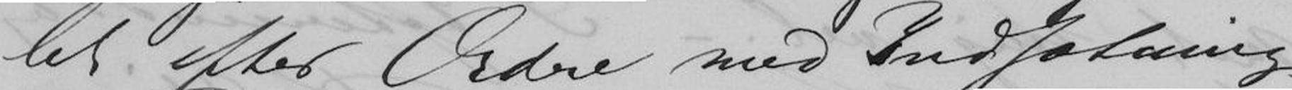let efter Ordre med Indsætning
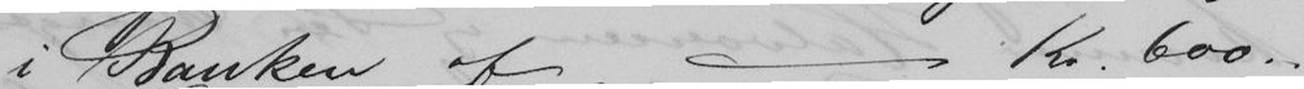i Banken af -------------Kr. 600..
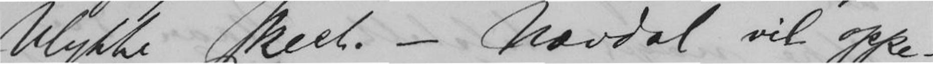Ulykke skeet. - Nævdal vil oppe-
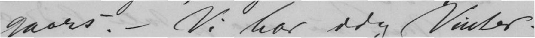gaars. - Vi har idag Vinter.
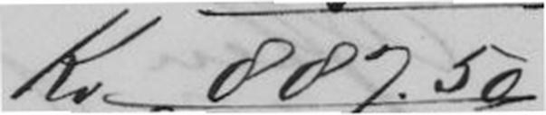Kr. 887.50
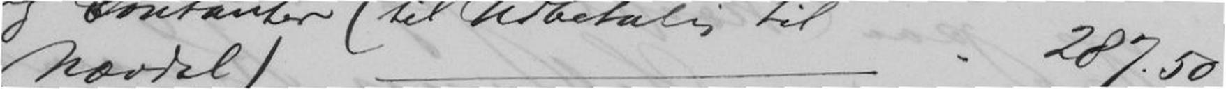Nævdal) ------------------ " 287.50
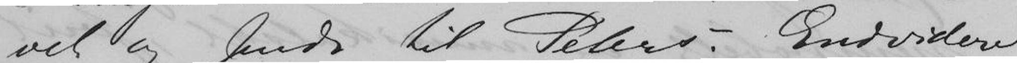vet og sendt til Peters. Endvidere
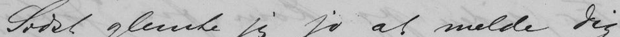Sidst glemte jeg jo at melde dig
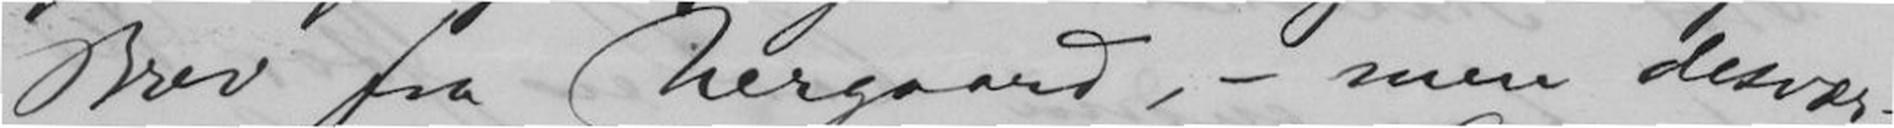Brev fra Nergaard, - men desvær-
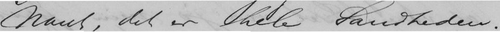Naut, det er hele Sandheden.
